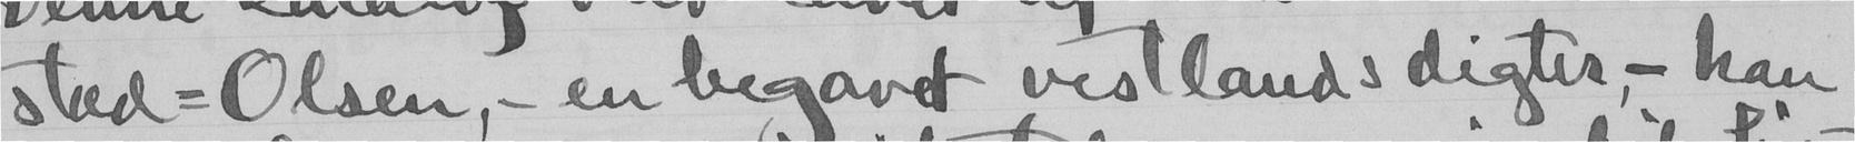stad-Olsen, - en begavet vestlands digter, - han
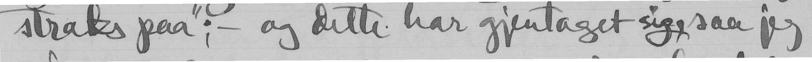straks paa"; - og dette har gjentaget sig, saa jeg
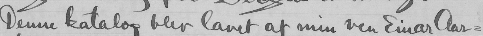Denne katalog blev lavet af min ven Einar Aar-
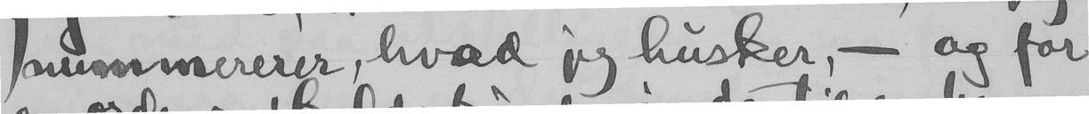nummererer, hvad jeg husker, - og for
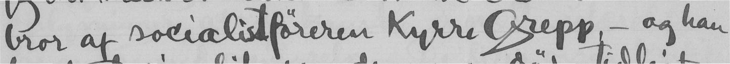bror af socialistføreren Kyrre Grepp - og han
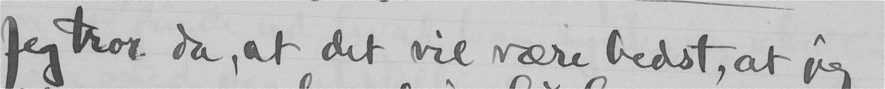Jeg tror da, at det vil være bedst, at jeg
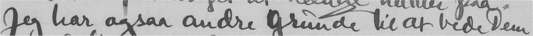Jeg har ogsaa andre grunde til at bede Dem
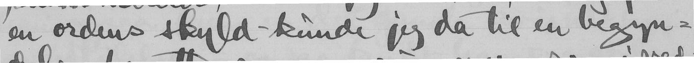en ordens skyld - kunde jeg da til en begyn-
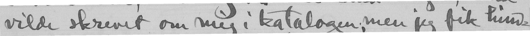vilde skrevet om mig i katalogen, men jeg fik hind-
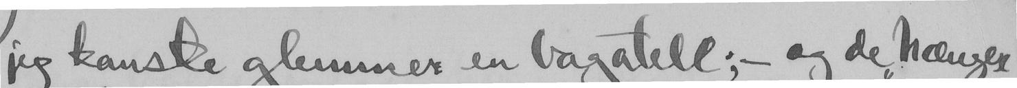jeg kanske glemmer en bagatell, - og de "hænger
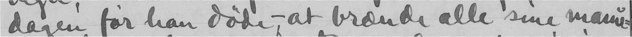dagen før han døde, at brænde alle sine manu-
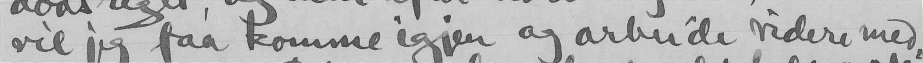vel jeg faa komme igjen og arbeide videre med
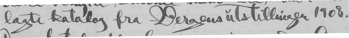lagte katalog fra Bergensutstillingen 1908.
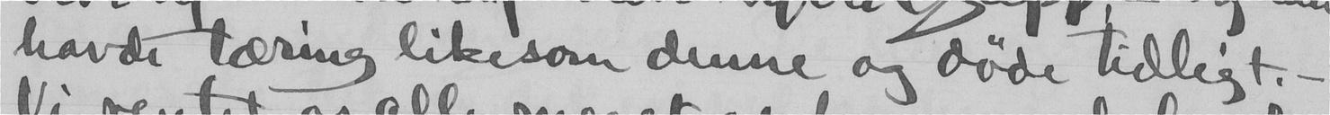havde tæring likesom denne og døde tidligt.-
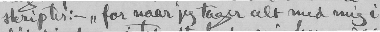skripter: - "for naar jeg tager alt med mig i
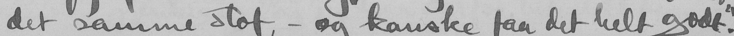det samme stof, - og kanske faa det helt godt."
